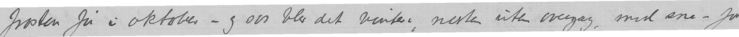frosten før i oktober – og saa blev det vinter, nesten uten overgang, med sne – for
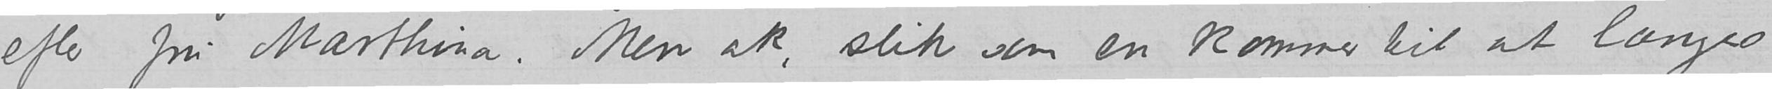efter fru Marthina. Men ak, slik som en kommer til at længes
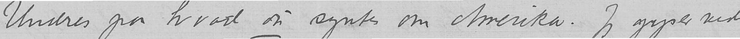Undres paa hvad du syntes om Amerika. Jeg gyser ved
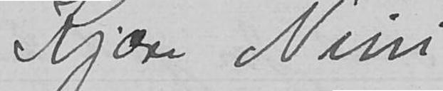Kjære Nini,
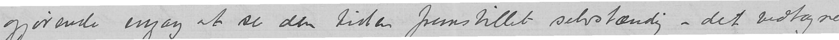gjørende engang at se den tiden fremstillet selvstændig – det vedtagne
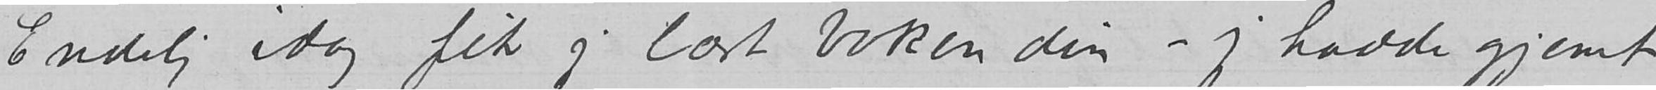Endelig idag fik jeg læst boken din – jeg hadde gjemt
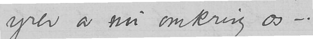yrer av nu omkring os -.
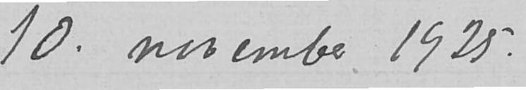10.november 1925
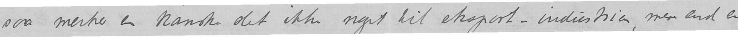saa merker en kanske det ikke noget til eksport-industrien, mere end en
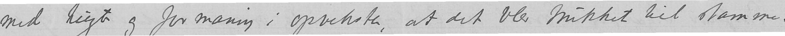med tugt og formaning i opveksten, at det blev trukket til skamme.
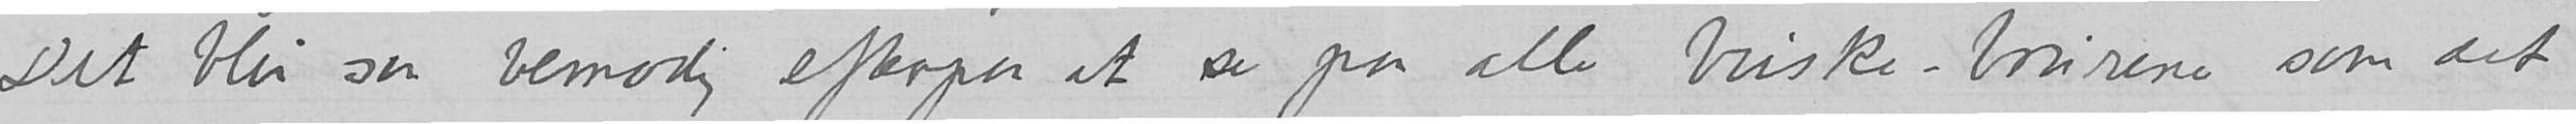Det blir saa vemodig efterpaa at se paa alle briske-brirerne som det
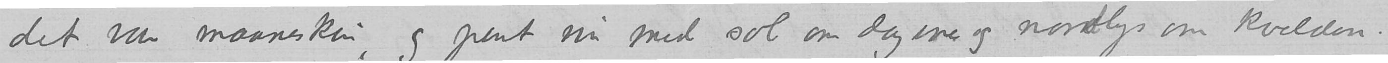det var maaneskin, og pent nu med sol om dagene og nordlys om kvelden.
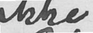ikke
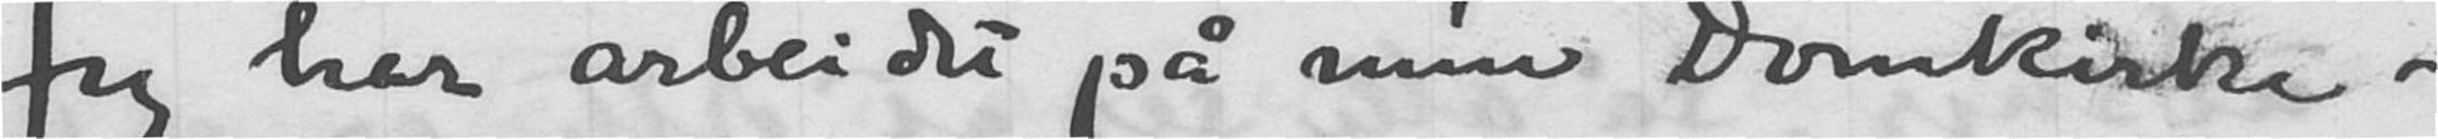Jeg har arbeidet på min Domkirke-
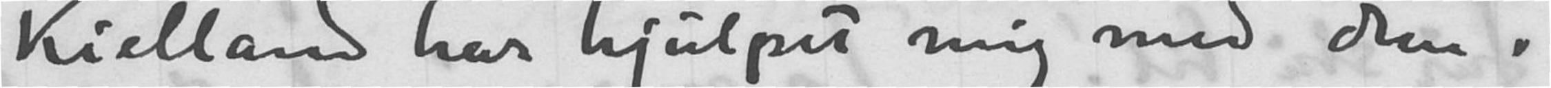Kielland har hjulpet mig med dem.
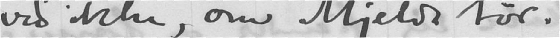ved ikke, om Mjelde tør.
